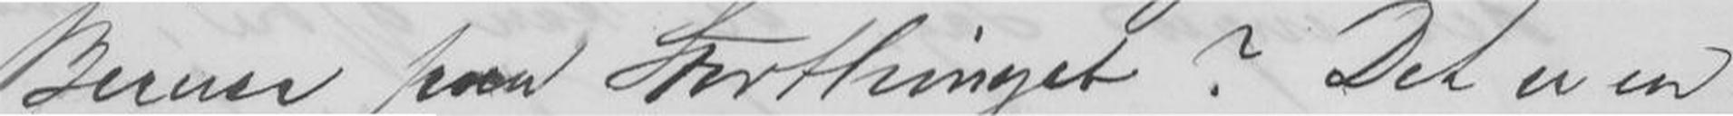Berner paa Storthinget? Det er en
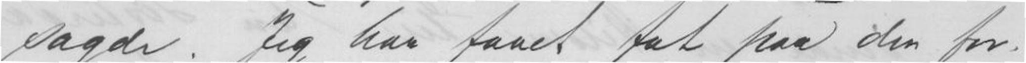sagde. Jeg har faaet fat paa den for-
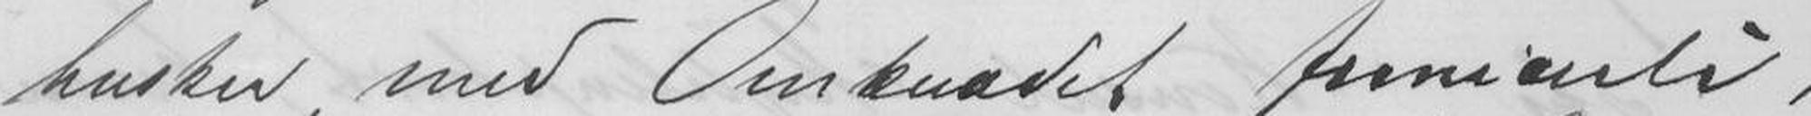husker, med Omkvædet funiculi,
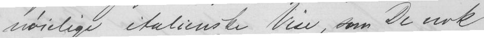nøielige italienske Vin, som De nok
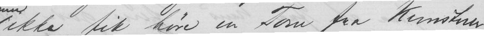ikke fik høre en Tone fra kunstner-
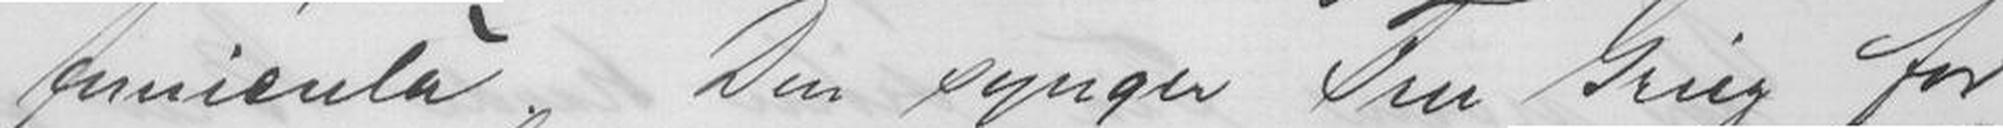funiculà. Den synger Fru Grieg for
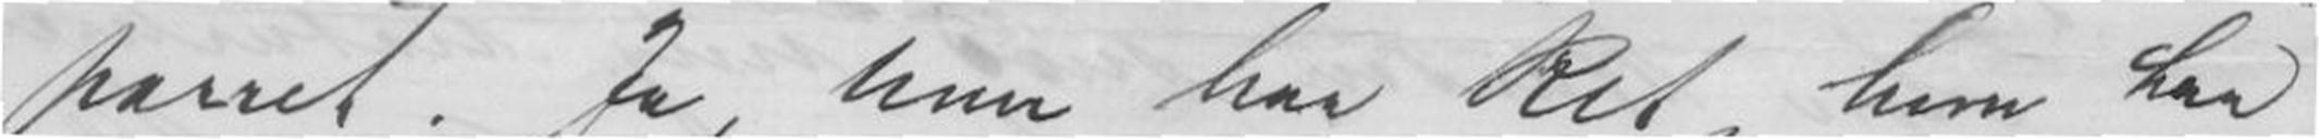parret. Ja, hun har Ret, hun har
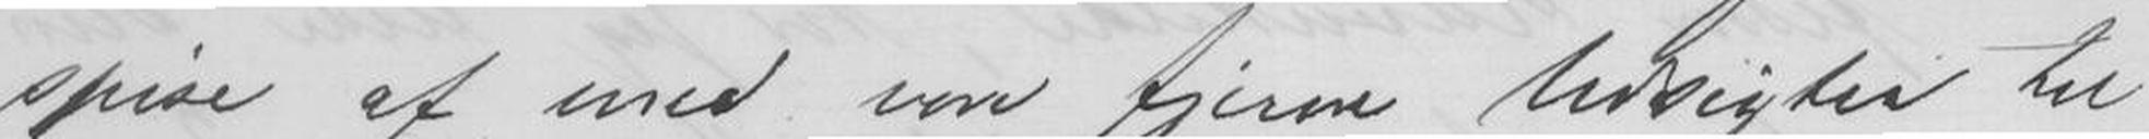spise af med vore fjerne Udsigter til
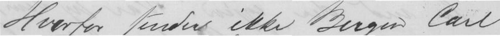Hvorfor sender ikke Bergen Carl
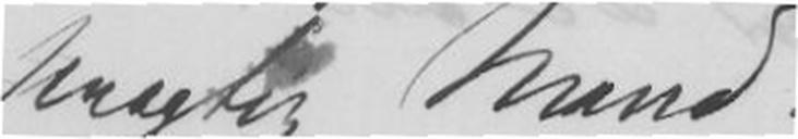prægtig Mand.
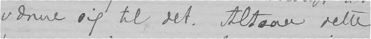vænne sig til det. Altsaa dette
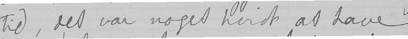tid, det var noget hvidt at have
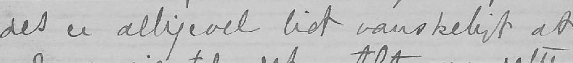det er alligevel lidt vanskeligt at
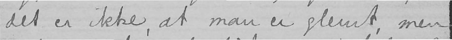det er ikke, at man er glemt, men
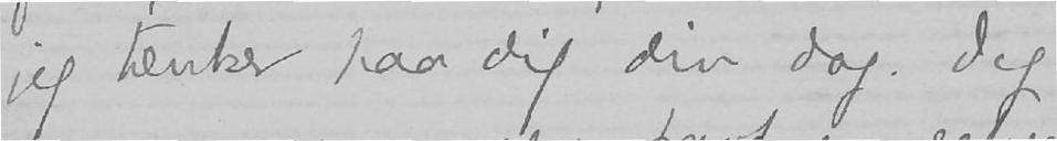jeg tænker paa dig din dag. Jeg
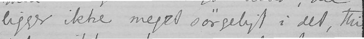ligger ikke meget sørgeligt i det, thi
